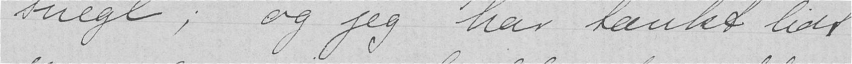snegl; og jeg har tænkt lidt
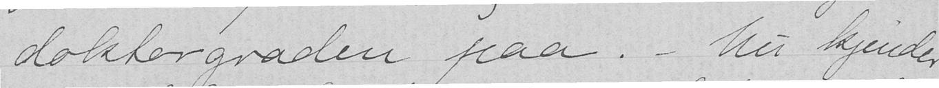doktorgraden paa. - Nu kjender
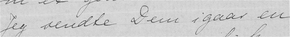Jeg sendte Dem igaar en
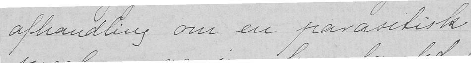afhandling om en parasitisk
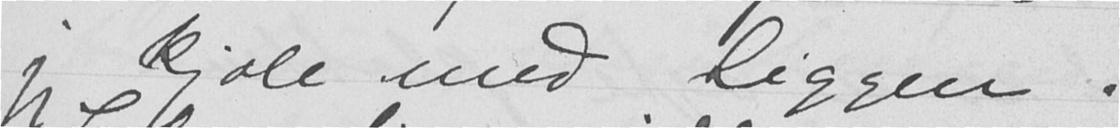jeg kjole med Siggen.
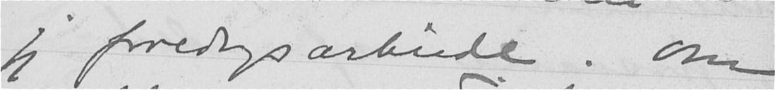jeg foredragsarbeide. Om
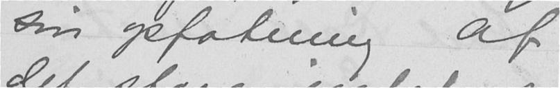sin opfatning af
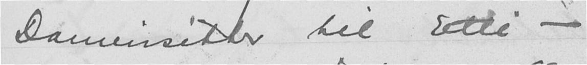Damevisitter til Etti -
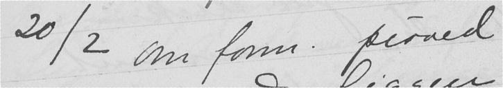20/2 Om form. prøved
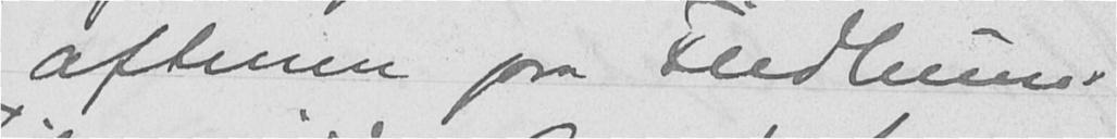aftenen paa Fridheim.
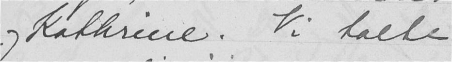og Kathrine. Vi talte
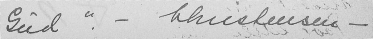Gud". - Christensen -
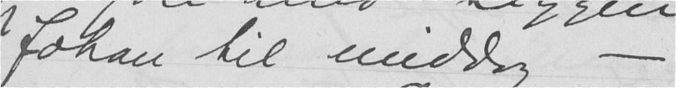Johan til middag -
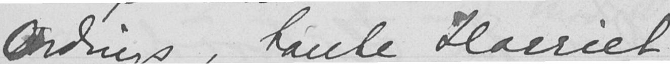Ordings, tante Harriet
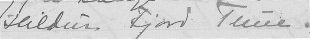Hildur Fjord Thue.
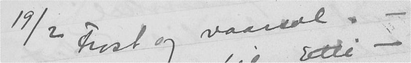19/2 Frost og vaarsol. -
